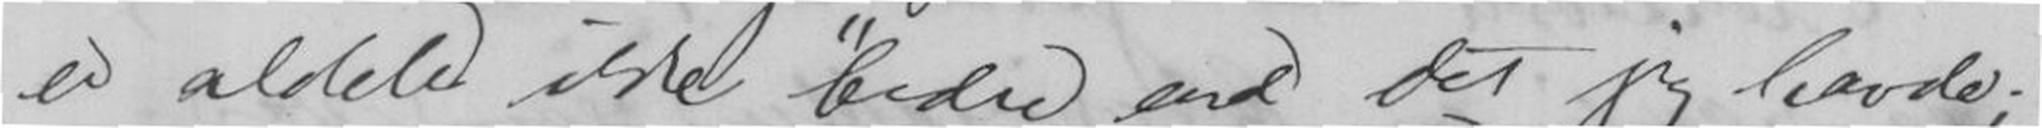er aldeles ikke bedre end det jeg havde;
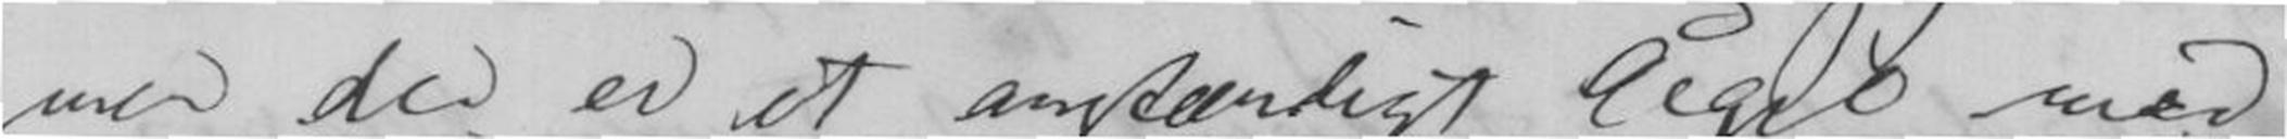men der er et anstændigt Orgel med
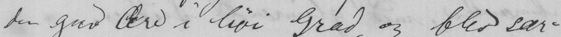den gav Ære i høi Grad, og blev sær-
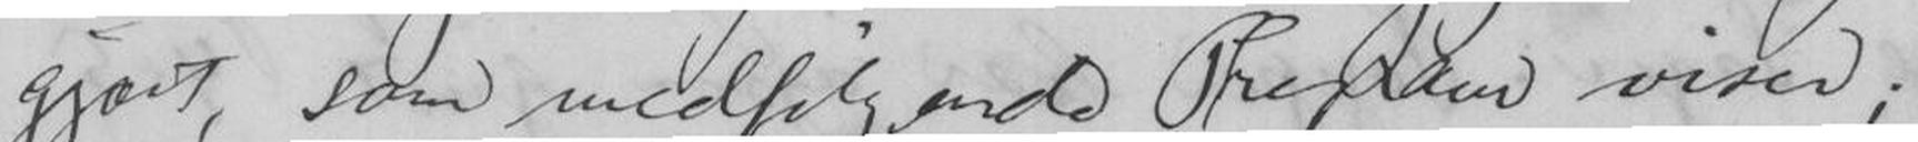gjort, som medfølgende Program viser;
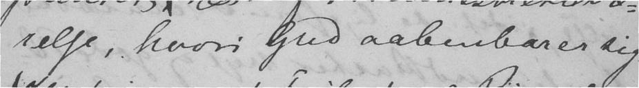relse, hvori Gud aabenbarer sig.
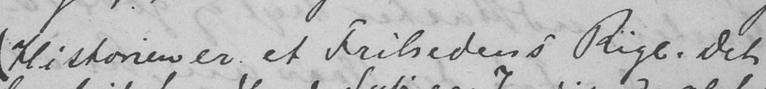(Historien er et Frihedens Rige. Det
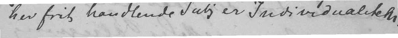her frit handlende Subj er Individualiteten.
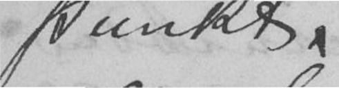punkt,
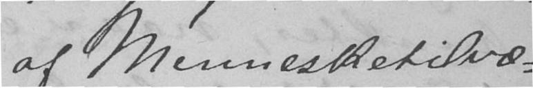af Mennesketilvæ-
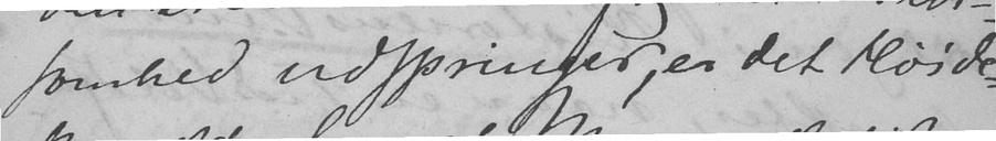somhed udspringer, er det Høide-
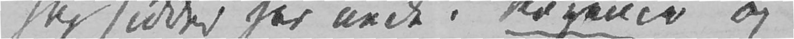Jeg sidder her nede i «Regence» og
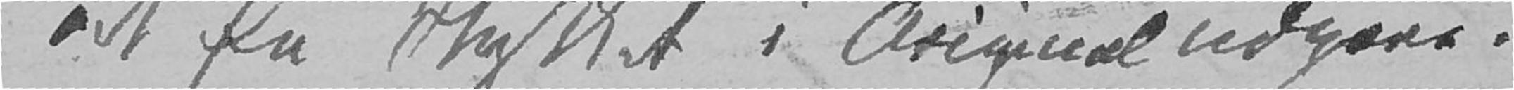at få Stykket i Originaludgave.
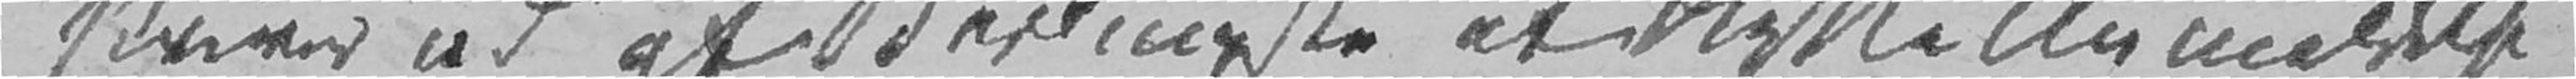skriver ud af Berlingske et Stykke Anmeldelse
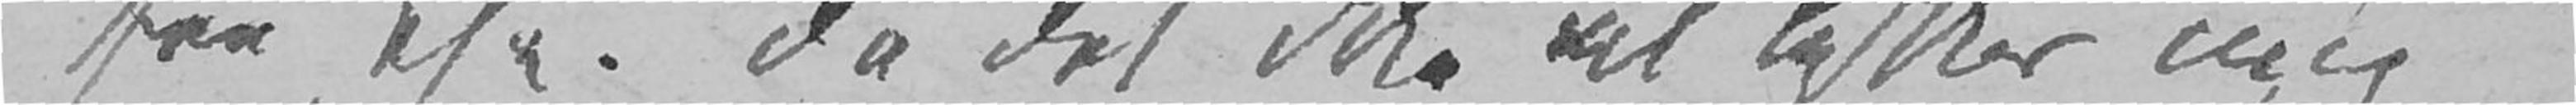fra Chr. da det ikke vil lykkes mig
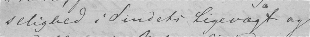selighed i Sindets Ligevægt og
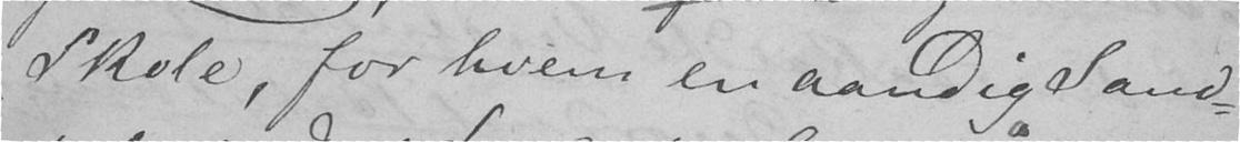Skole, for hvem en aandig Sand-
